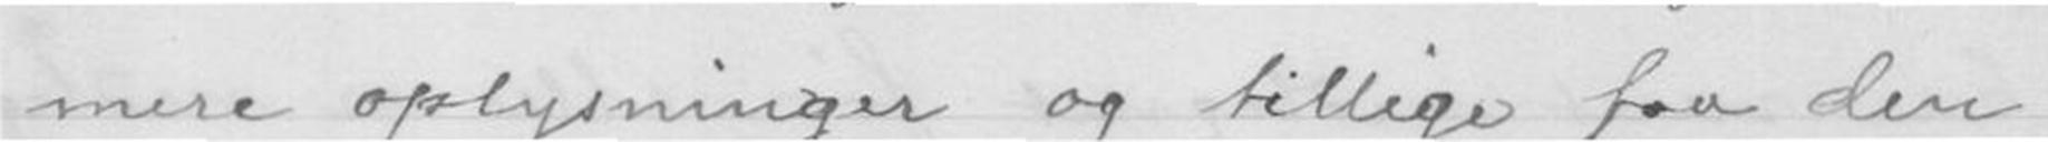mere oplysninger og tillige faa den
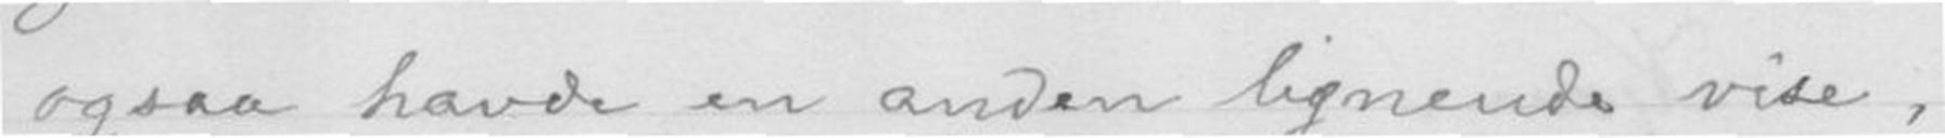ogsaa havde en anden lignende vise,
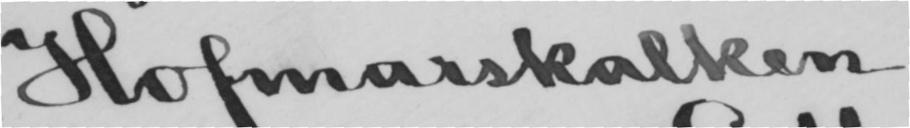Hofmarskalken
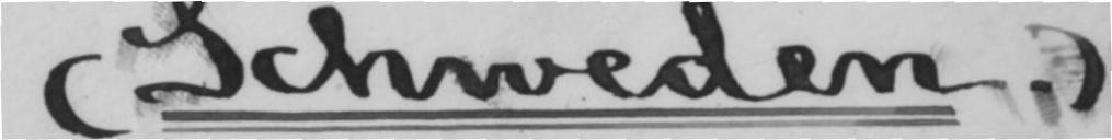(Schweden.)
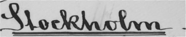Stockholm.
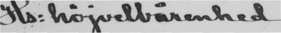Hs: højvelbårenhed
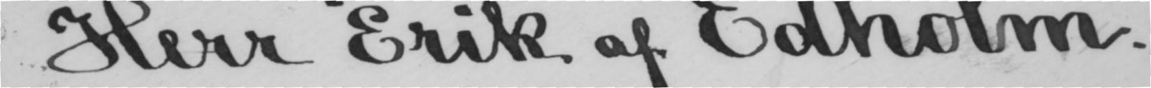Herr Erik af Edholm.
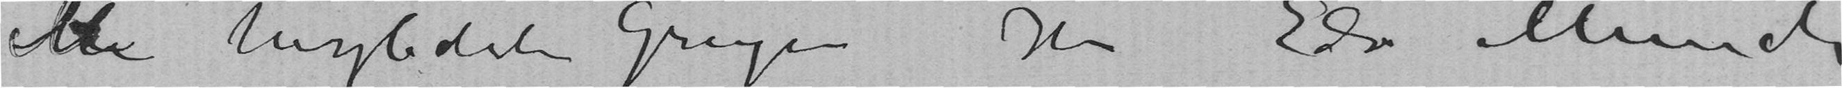Mi herzlichste Gruszen Ihr Edv Munch
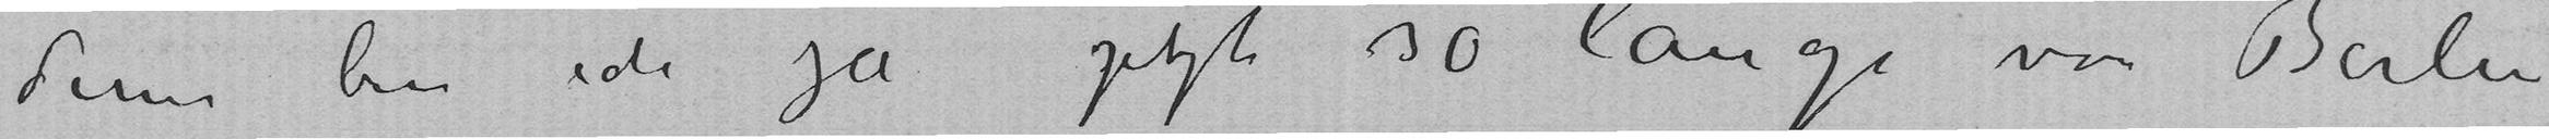denn bin ich ja jetzt so lange von Berlin
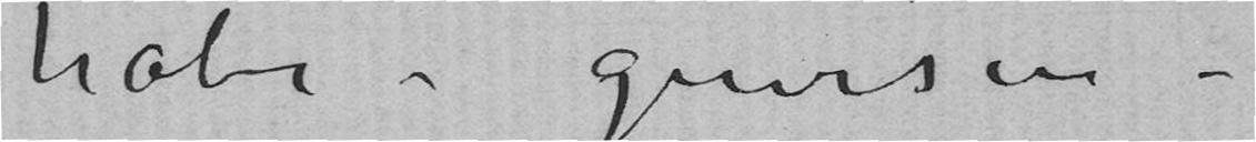habe – gewesen –
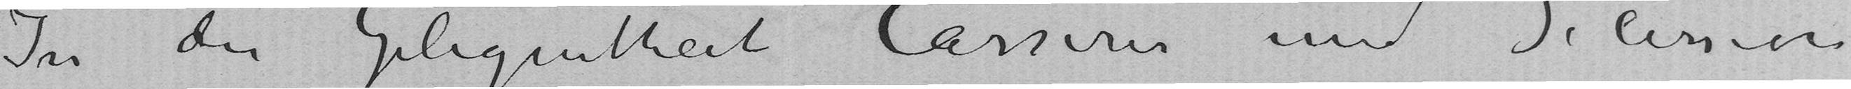In der Gelegentheit Cassirer und Secession
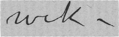wek –
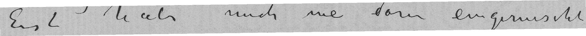Erst habe mich nie darin eingemischt
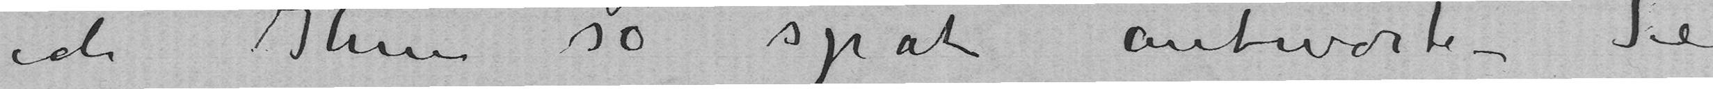ich Ihm so spat antworten Sie
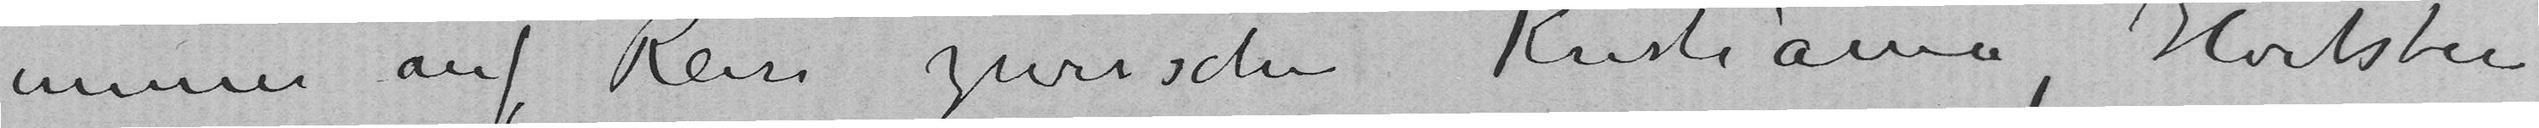immer auf Reise zwischen Kristiania, Hvitsten
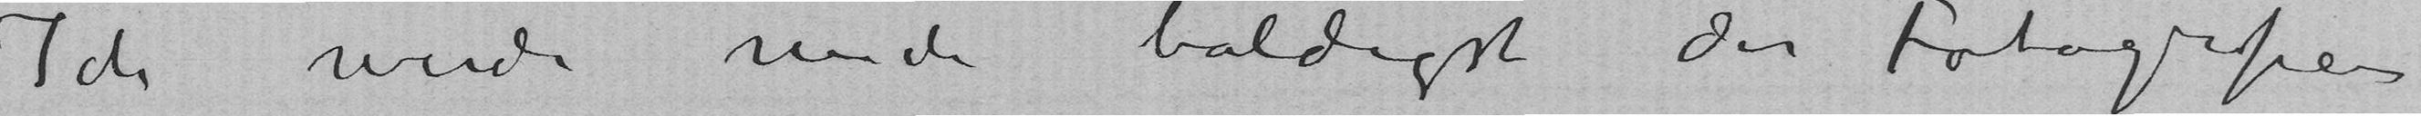Ich werde mich baldigst der Fotografien
